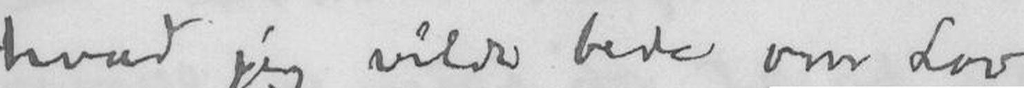hvad jeg vilde bede om Lov
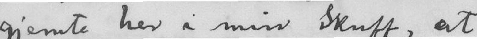gjemte her i min Skuff, at
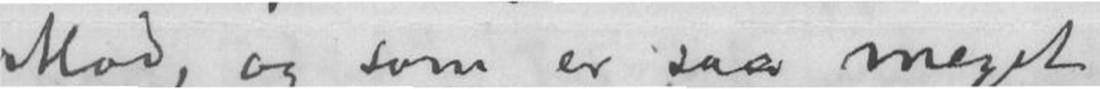Mod, og som er saa meget
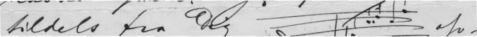tildels fra Dig
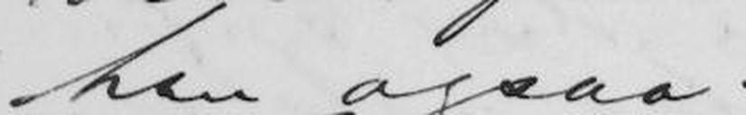han ogsaa!
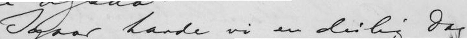Igaar havde vi en deilig Dag
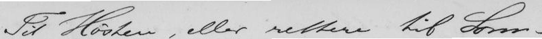Til Høsten, eller rettere til Som-
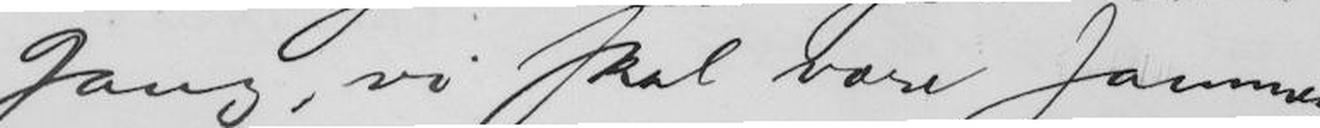Gang, vi skal være sammen!
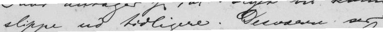slippe ud tidligere. Desværre ser
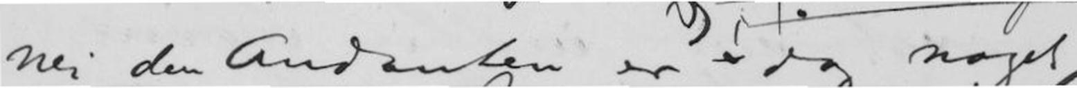nei den Andanten er dog noget
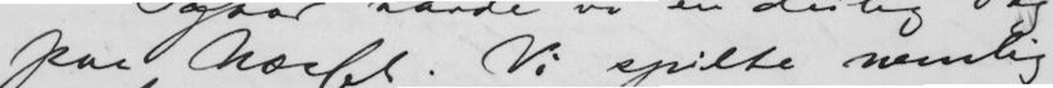paa Næsset. Vi spilte nemlig
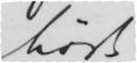hørt Noter
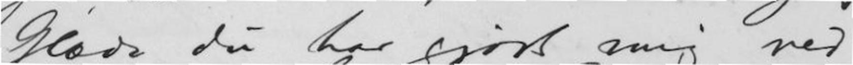Glæde Du har gjort mig ved
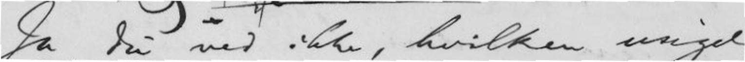Ja Du ved ikke, hvilken usigelig
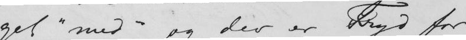get "med" og der er Fryd for
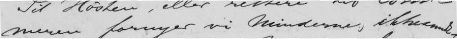meren fornyer vi Minderne, ikkesandt?
